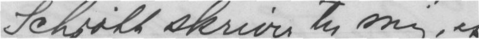Schjøtt skriver til mig, at
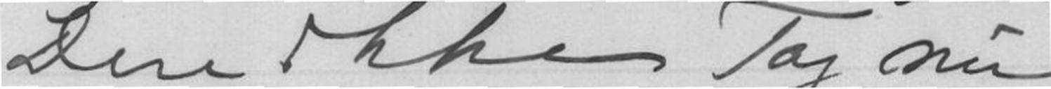Dere ikke. Tag nu
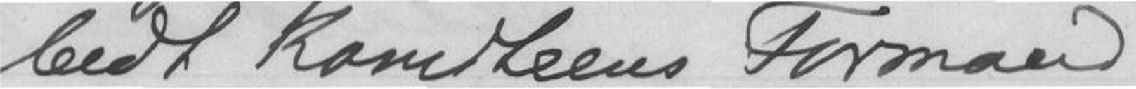bedt Komiteens Formand
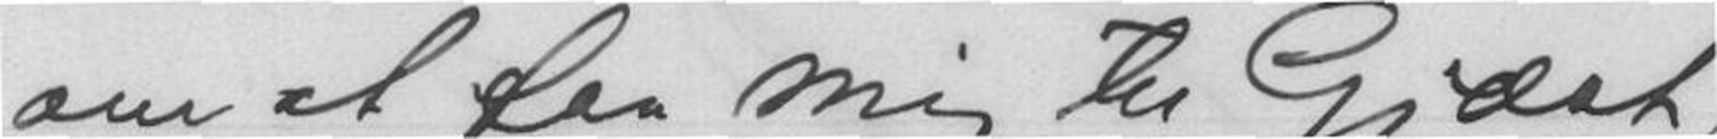om at faa mig til Gjæst
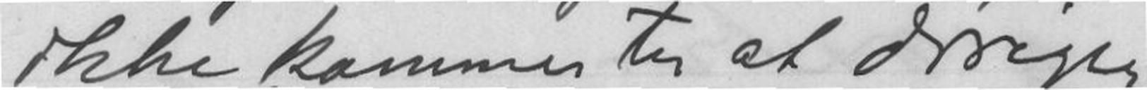ikke kommer til at dirigere.
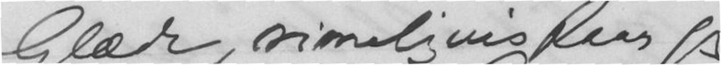Glæde, rineligvis faar jeg
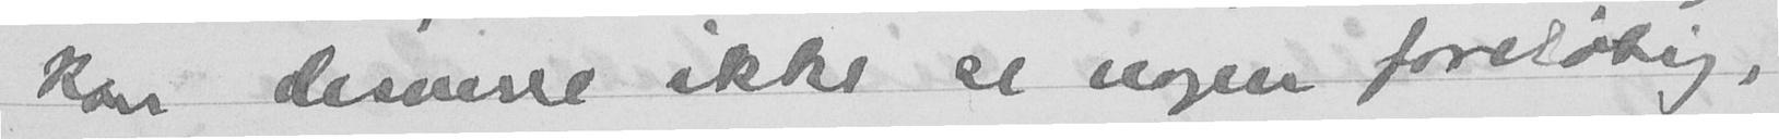kan dessverre ikke se nogen foreløbig,
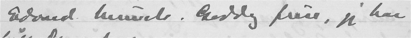Edvard Munch. Goddag frue, jeg har
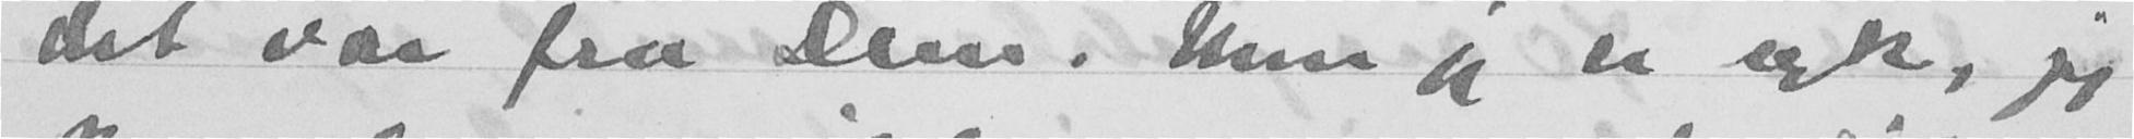det var fra Dem. Men jeg er syk, jeg
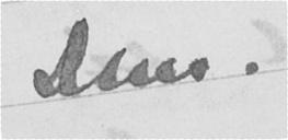Dem.
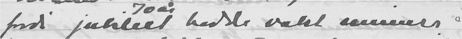fordi jubileet 70 år hadde vakt minner i
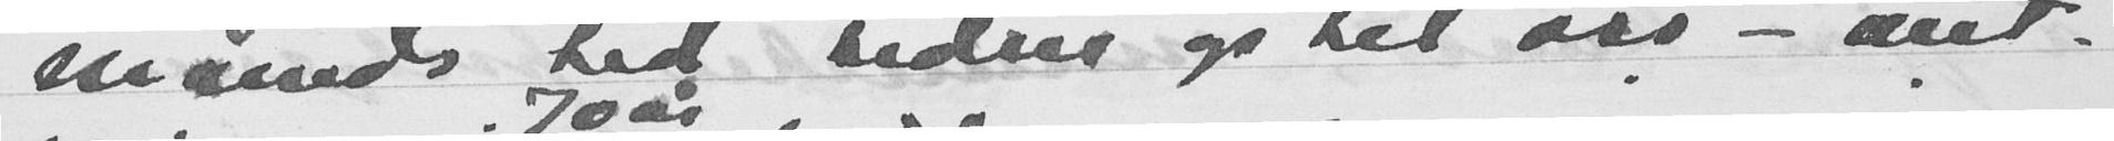måneds tid siden op til oss - ant.
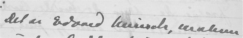Det er Edvard Munch, maleren
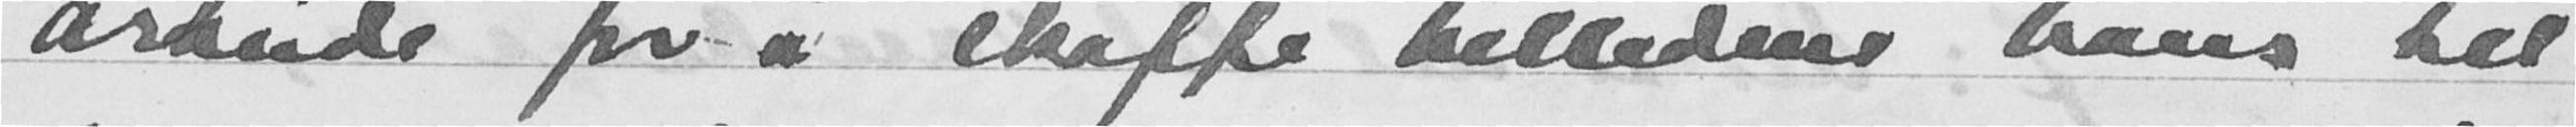arbeide for skaffe billedene hans til
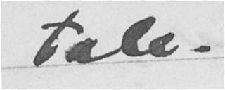tale.
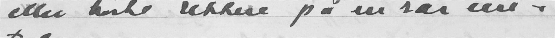eller hørte rettere på en rar sam-
